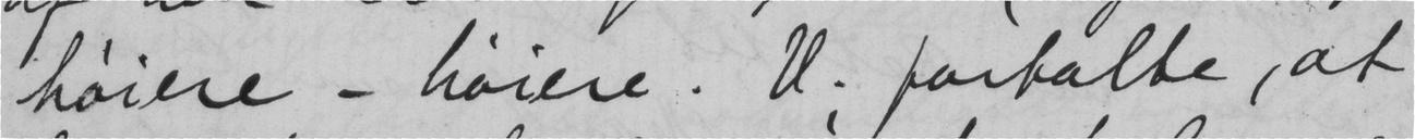høiere - høiere. V. fortalte, at
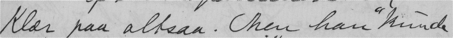Klær paa altsaa. Men han "kunde
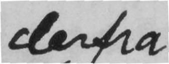derfra
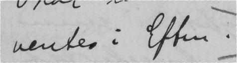ventes i Efter.
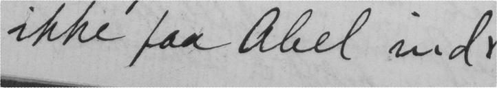ikke faa Abel ind
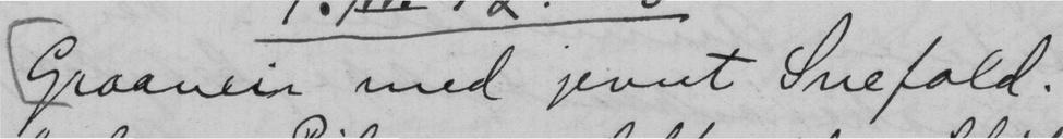Graaveir med jevnt Snefald.
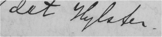det Hylster.
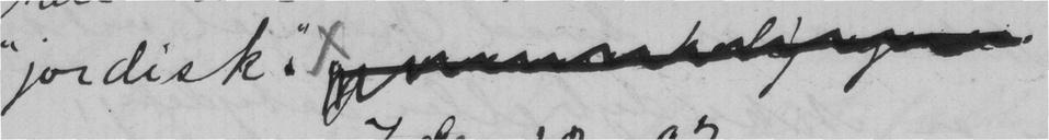"jordisk". x
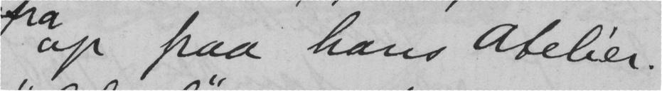op paa hans Atelier.
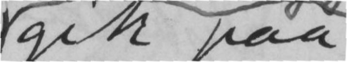gik paa
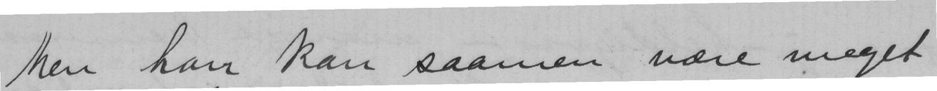Men han kan saamen være meget
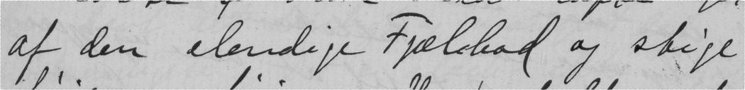af den elendige Fjælebad
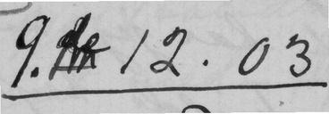9. 12.03
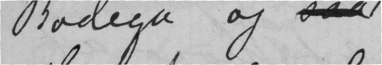Bodega og saa
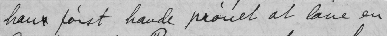han først havde prøvet at lave en
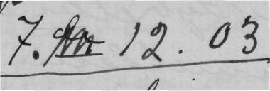7. 12.03
